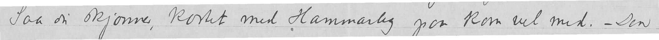Saa du skjønner, kortet med Hammarby paa kom vel med. – Den
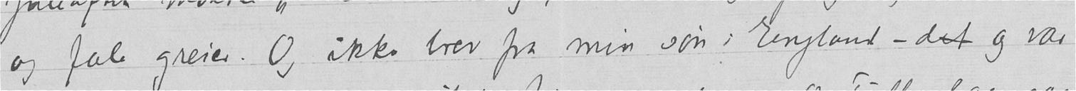og fæle greier. Og ikke brev fra min søn i England – det og var
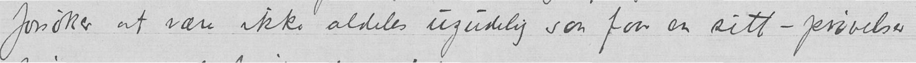forsøker at være ikke aldeles ugudelig saa faar en sitt – prøvelser
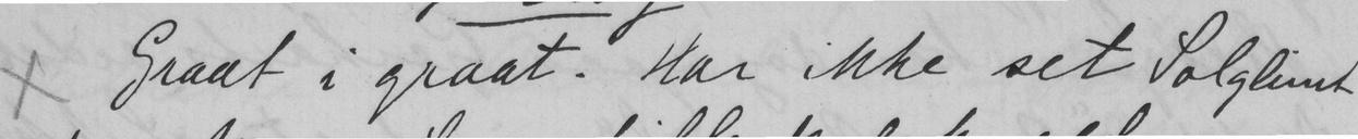x Graat i graat. Har ikke set Solglimt
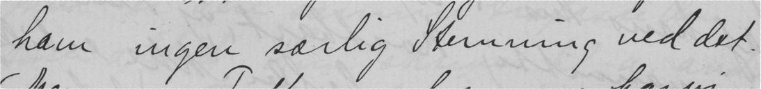ham ingen særlig stemning ved det.
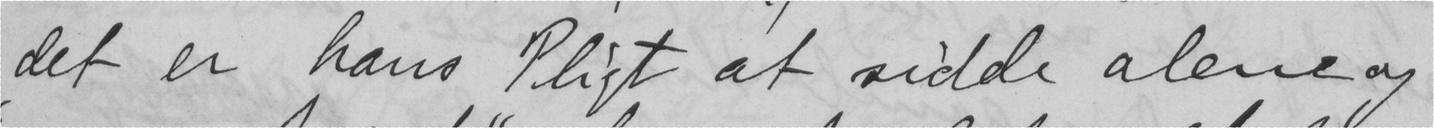det er hans Pligt at sidde alene og
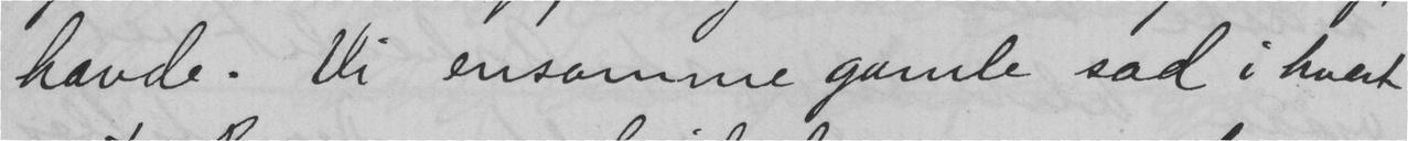havde. Vi ensomme gamle sad i hvert
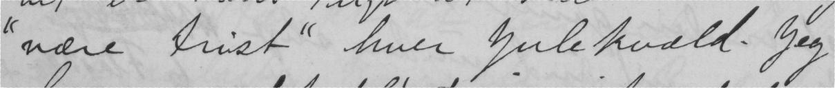"være trist" hver julekvæld. Jeg
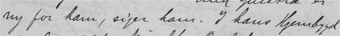ny for ham, siger han. I hans Hjembygd
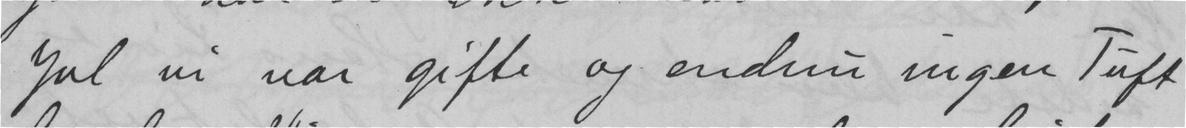Jul vi var gifte og endnu ingen Tuft
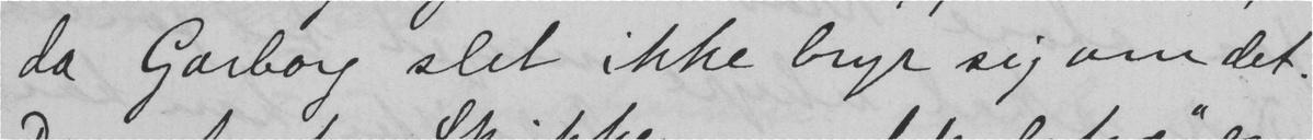da Garborg slet ikke bryr sig om det.
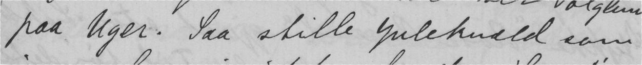paa Uger. Saa stille Julekvæld som
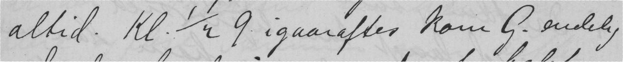altid. Kl. 1/2 9. igaaraftes kom G. endelig
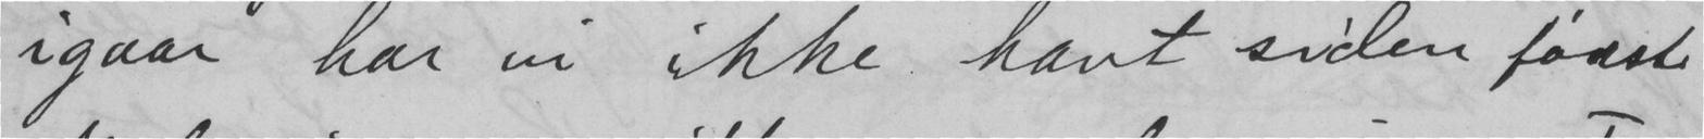igaar har vi ikke havt siden første
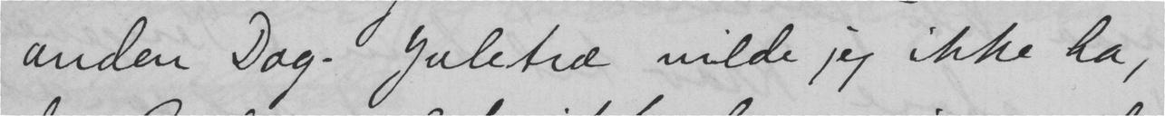anden Dag. Juletræ vilde jeg ikke ha,
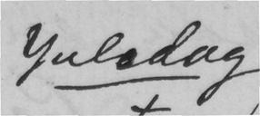Juledag
